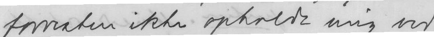forresten ikke opholde mig ved'
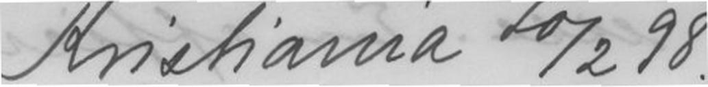Kristiania 10/2 98
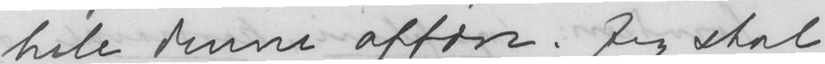hele denne affære. Jeg skal
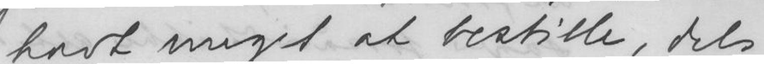havt meget at bestille, dels
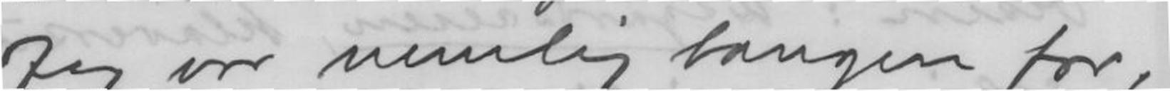Jeg var nemlig bangen for,
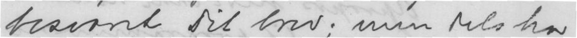besvaret dit brev; men dels har
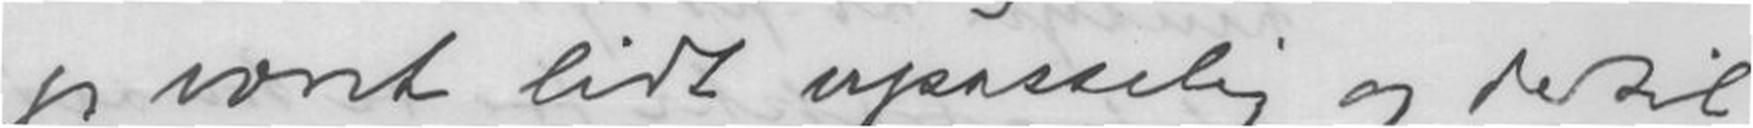jeg været lidt upasselig og dertil
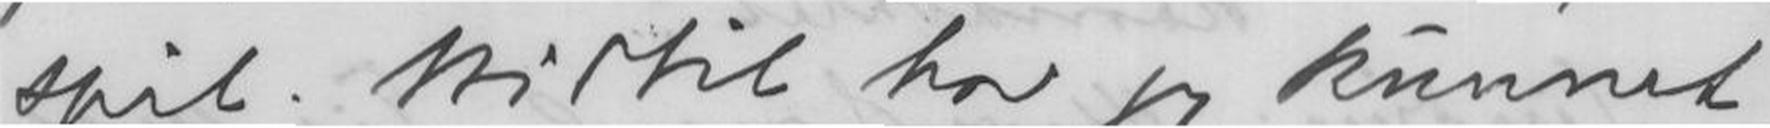spil. Hidtil har jeg kunnet
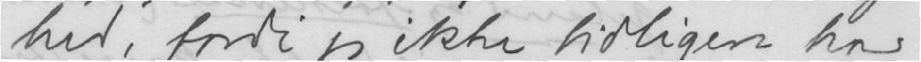hed, fordi jeg ikke tidligere har
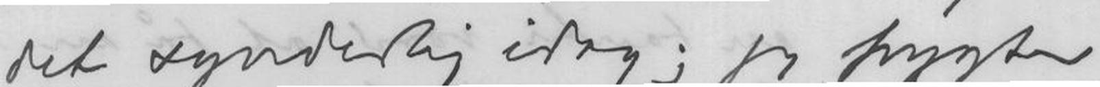det synderlig idag; jeg frygter
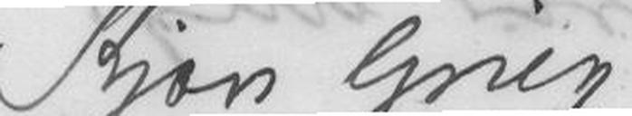Kjære Grieg!
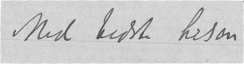Med bedste hilsen
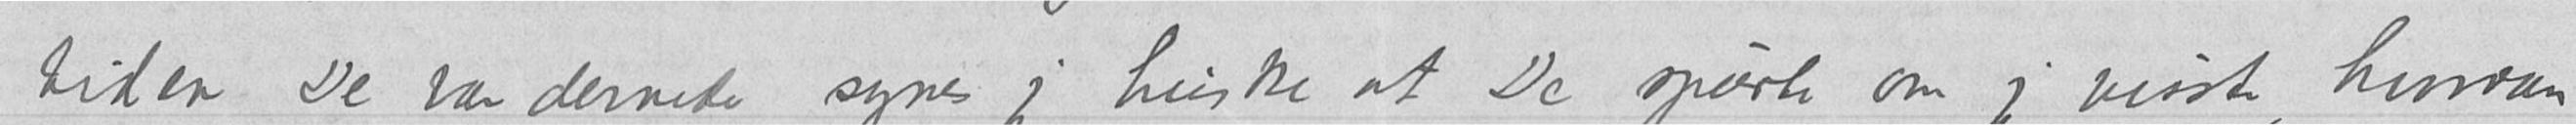tiden De var dernede synes jeg huske at De spurte om jeg visste, hvordan
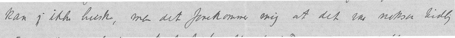kan jeg ikke huske, men det forekommer mig at det var noksaa tidlig
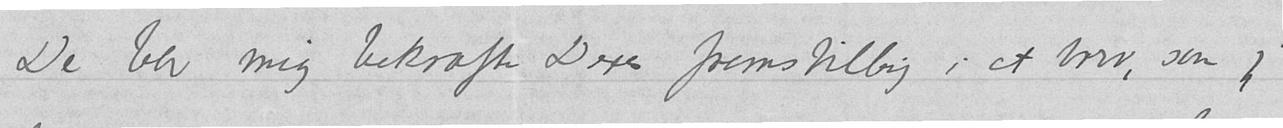De ber mig bekræfte Deres fremstilling i et brev, som jeg
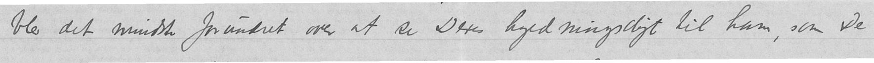blev det mindste forundret over at se Deres hyldningsdigt til ham, som De
